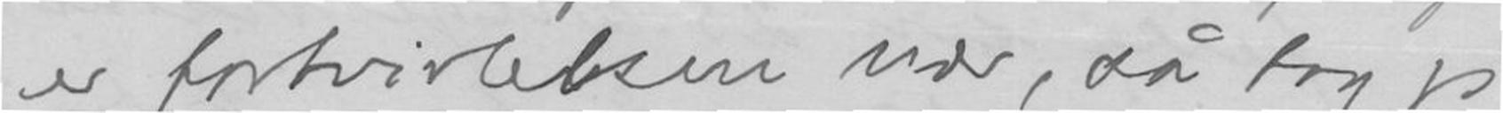er fortvilelsen nær, så tog jeg
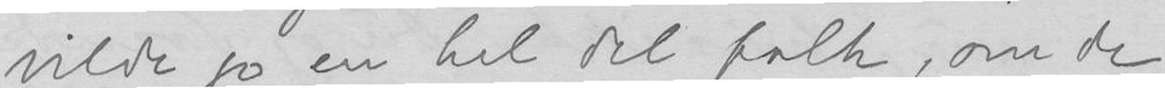vilde jo en hel del folk, om de
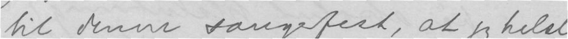til denne sangefest, at jeg helst
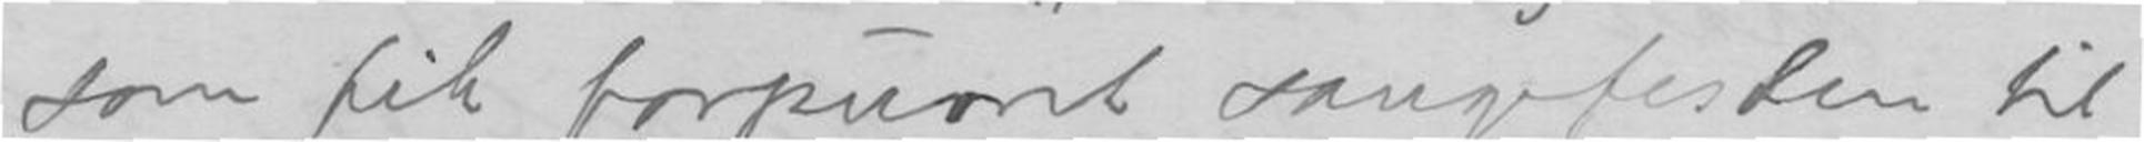som fik forpurret sangerfesten til
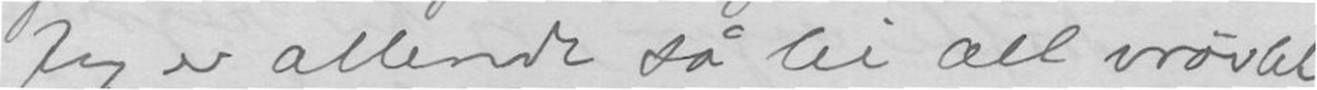Jeg er allerede så lei alt vrøvlet
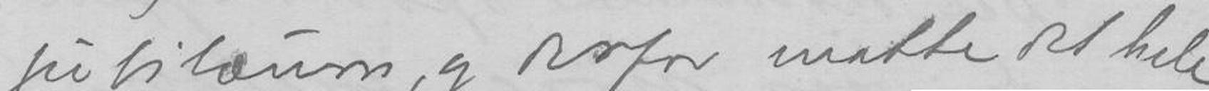jubilæum, og derfor måtte det hele
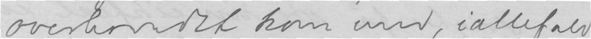overhovedet kom med, iallefald
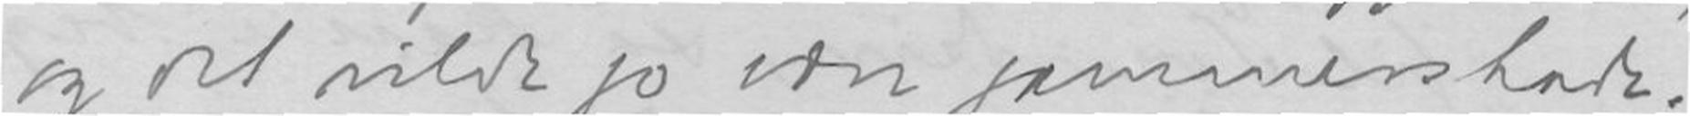og det vilde jo være jammershode.
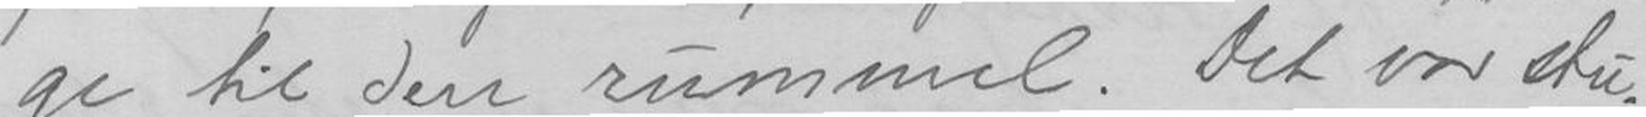ge til den rummel. Det var stu-
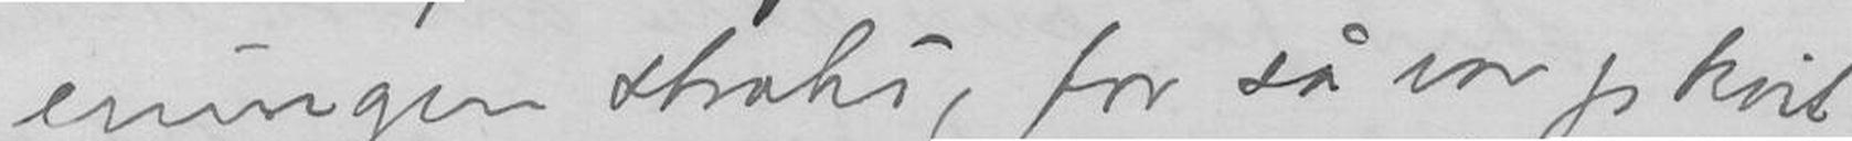eningen straks, for så var jeg kvit
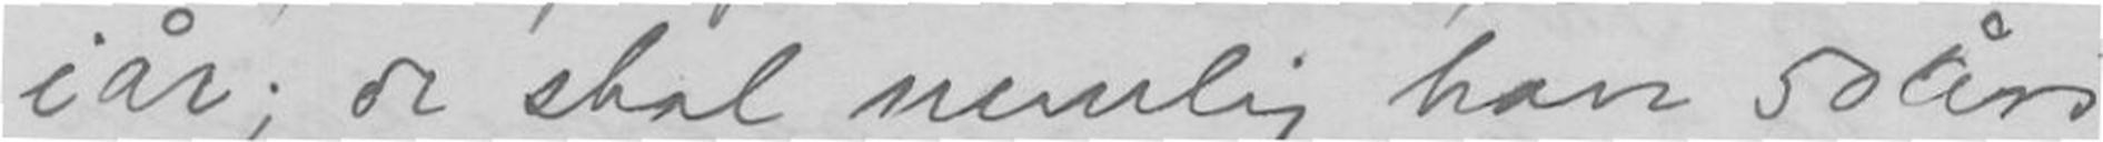iår; de skal nemlig have 50 års
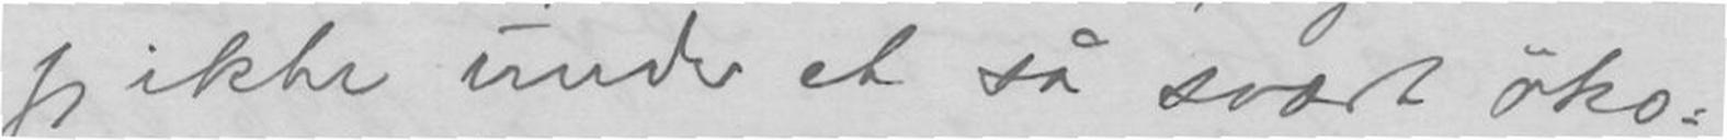jeg ikke under et så svært øko-
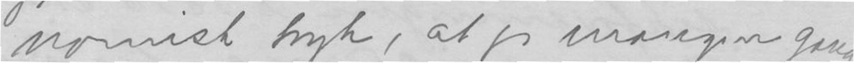nomisk tryk, at jeg mangen gang
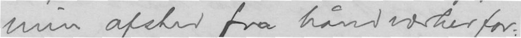min afsted fra håndværkerfor-
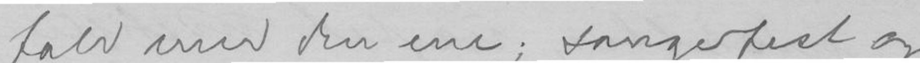fald med den ene; sangerfest og
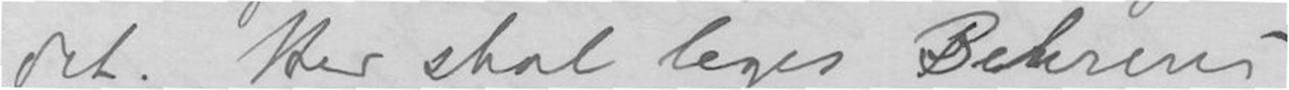det. Her skal leges Bekrenes
